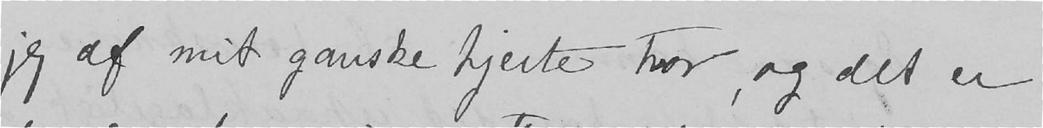jeg af mit ganske hjerte tror, og det er
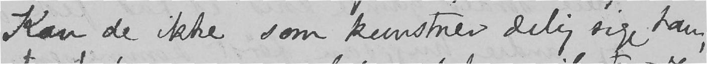Kan de ikke som kunstner ærlig sige ham,
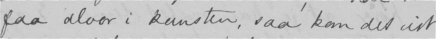faa alvor i kunsten, saa kom det vist
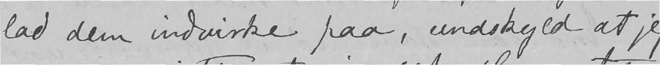lad dem indvirke paa, undskyld at jeg
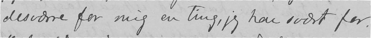desværre for mig en ting, jeg har svært for.
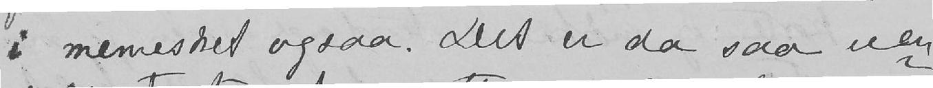i mennesket ogsaa. Det er da saa uen
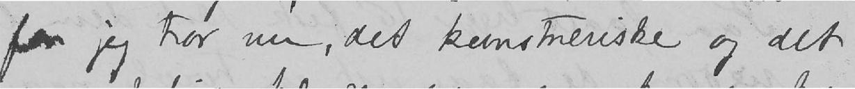for jeg tror nu, det kunstneriske og det
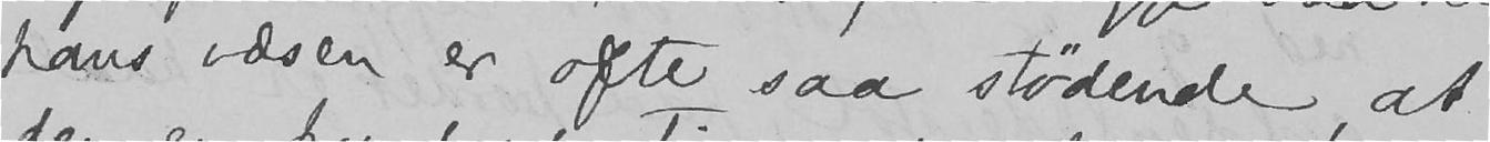hans væsen er ofte saa stødende, at
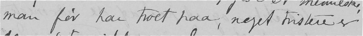man før har troet paa, noget tristere er
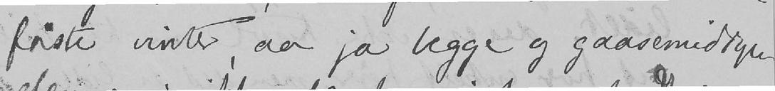første vinter, aa ja begge og gaasemiddager
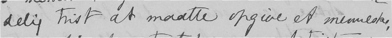delig trist at maatte opgive et menneske,
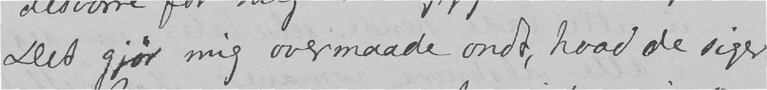Det gjør mig overmaade ondt, hvad de siger
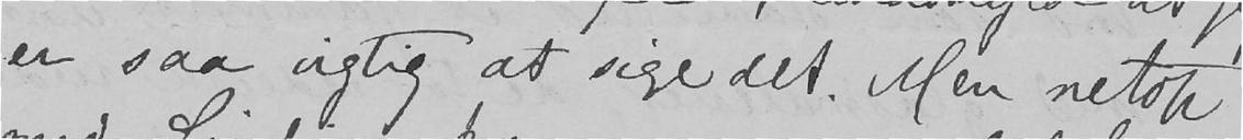er saa vigtig at sige det. Men netop
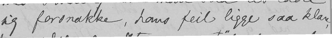sig forsnakke, hans feil ligge saa klare,
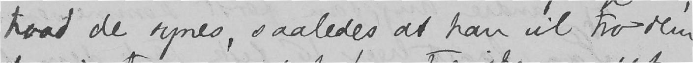hvad de synes, saaledes at han vil tro dem
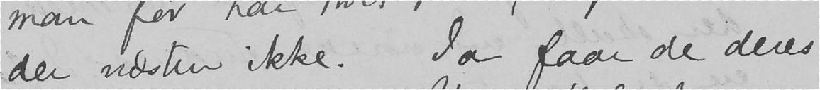der næsten ikke. Ja faar de deres
